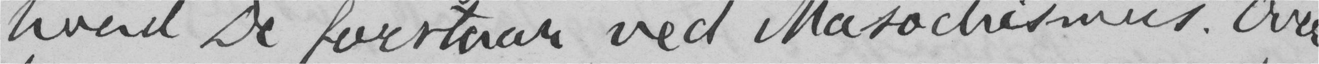hvad De forstaar ved Masochismus. Over-
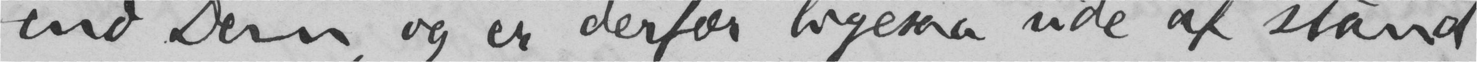end Dem, og er derfor ligesaa ude af stand
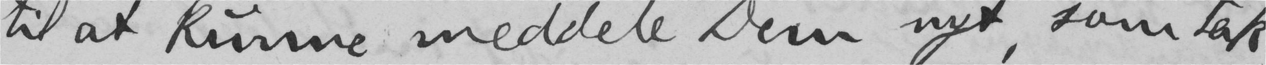til at kunne meddele Dem nyt, som tak-
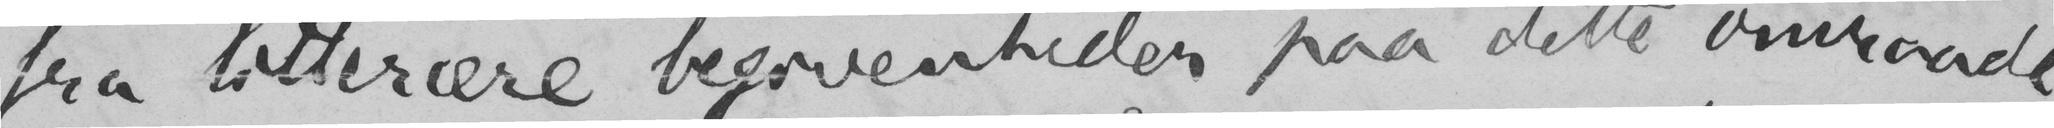fra litterære begivenheder paa dette omraade
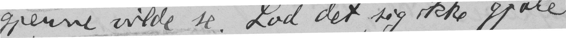gjerne vilde se. Lod det sig ikke gjøre
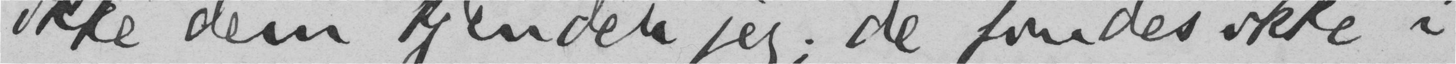ikke dem kjender jeg; de findes ikke i
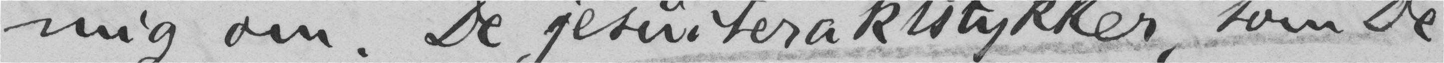mig om. De jesuiteraktstykker, som De
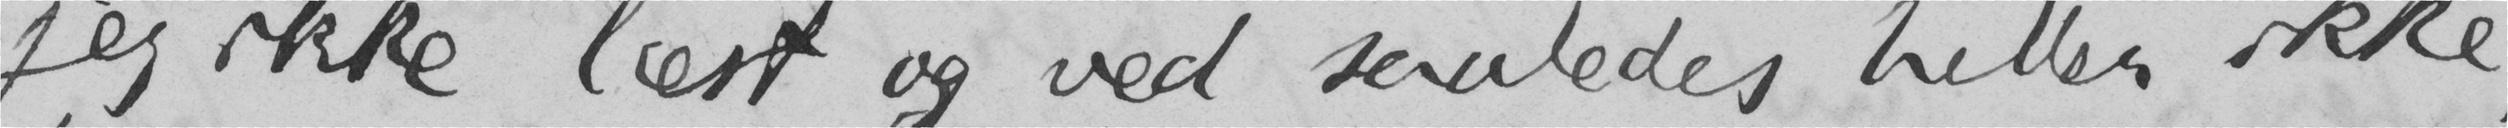jeg ikke læst og ved saaledes heller ikke,
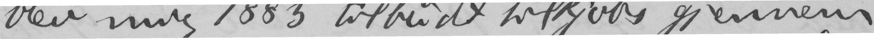blev mig 1883 tilbudt tilkjøbs gjennem
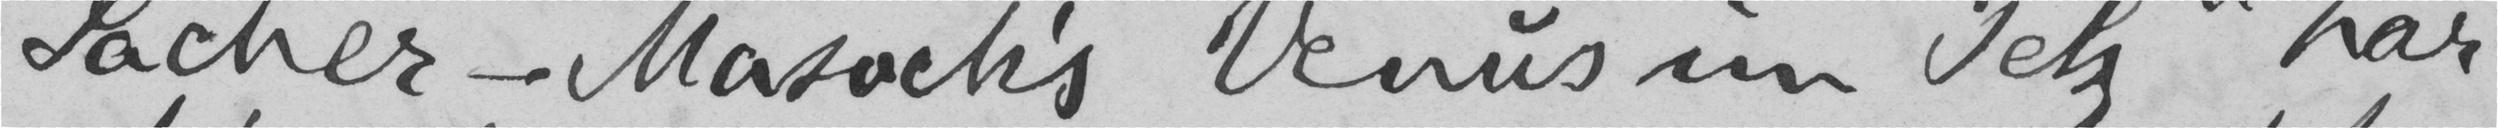Sacher-Masoch’s «Venus im Pelz» har
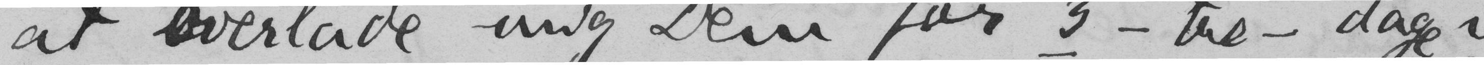at overlade mig Dem for 3 - tre - dage?
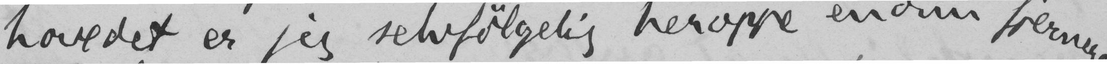hovedet er jeg selvfølgelig heroppe endnu fjernere
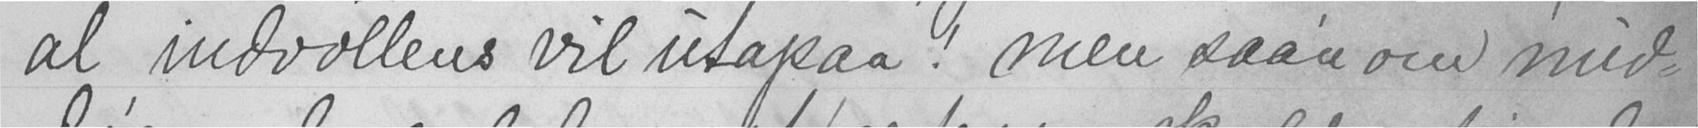al indvollens vil utapaa! men saa'n om mid-
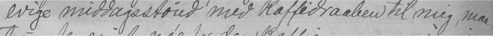evige middagsstønd med kaffedraaben til mig, maa
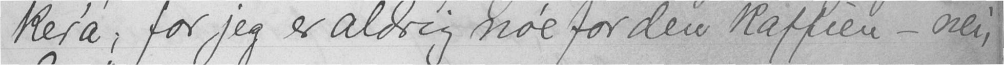ker'a; for jeg er aldrig no'e for den kaffien - nei,
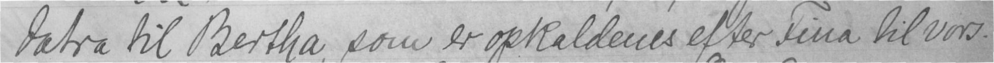datra til Bertha, som er opkaldenes efter Tina til vors.
